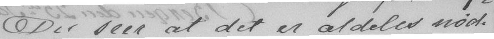Du seer at det er aldeles nød-
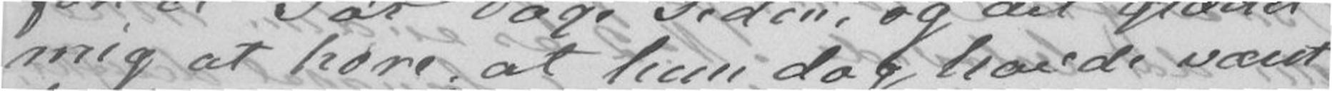mig at høre at hun dog havde været
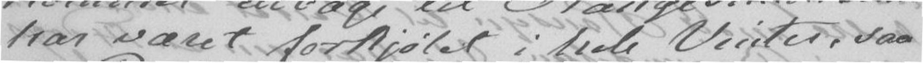har været forkjølet i hele Vinter, saa
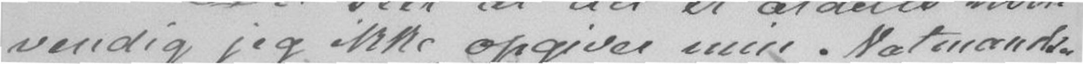vendig jeg ikke opgiver min Natmands-
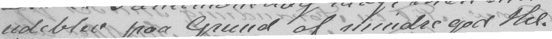udeblev paa Grund af mindre god Hel-
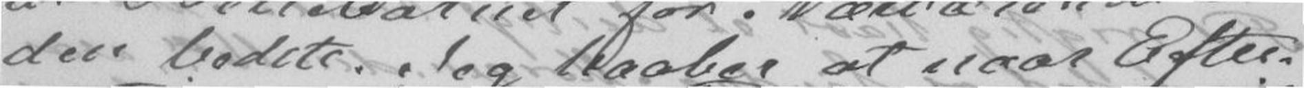den bedste. Jeg haaber at naar Efter-
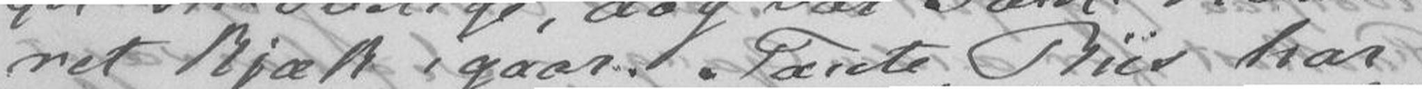ret kjæk igaar. Tante Riis har
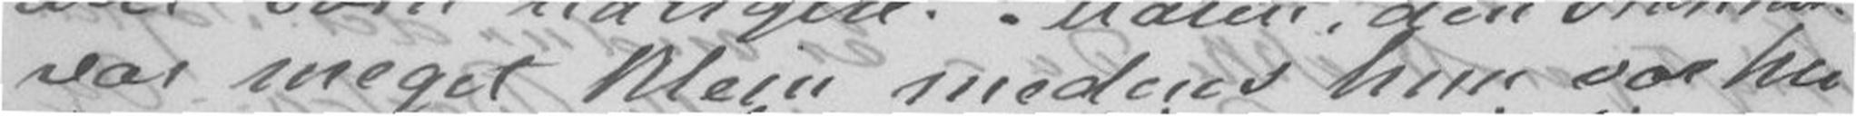var meget klein medens hun var her
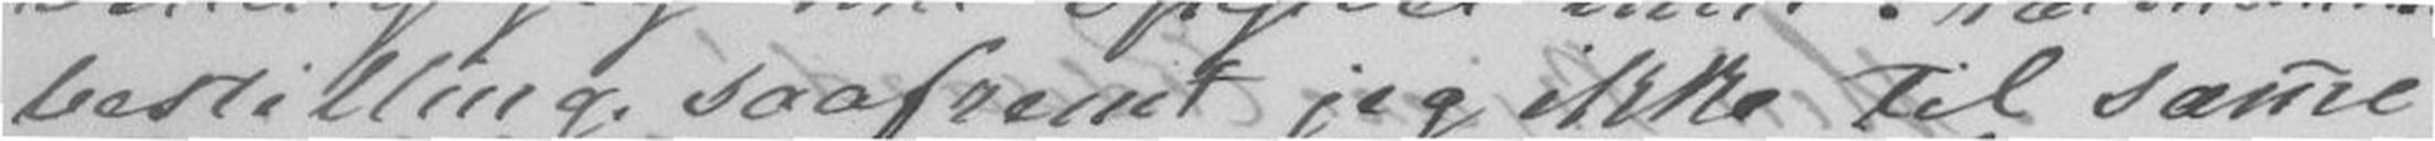bestilling saafremt jeg ikke til sa`me
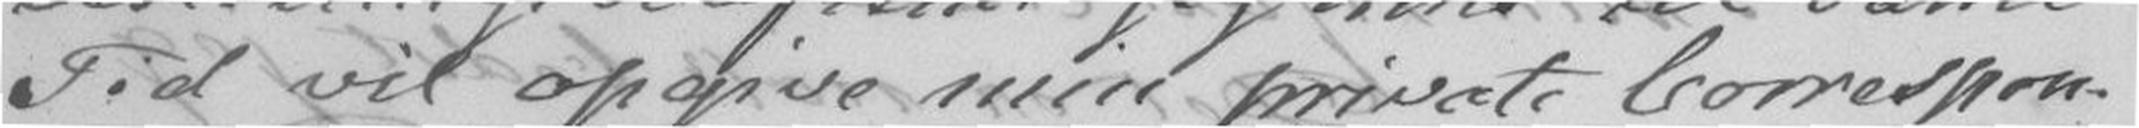Tid vil opgive min private Correspon-
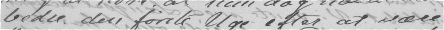bedre den første Uge efter at være
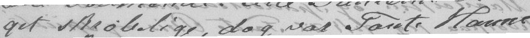get skrøbelig, dog var Tante Hanne
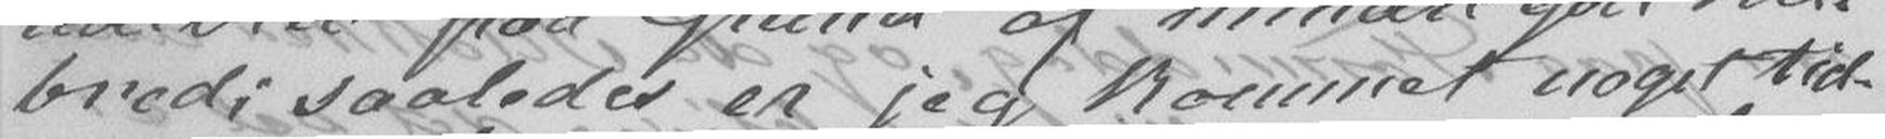bred; saaledes er jeg kommet noget tid-
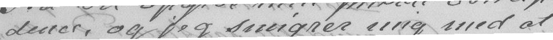dence, og jeg smigrer mig med at
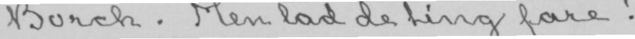Borch. Men lad de ting fare!
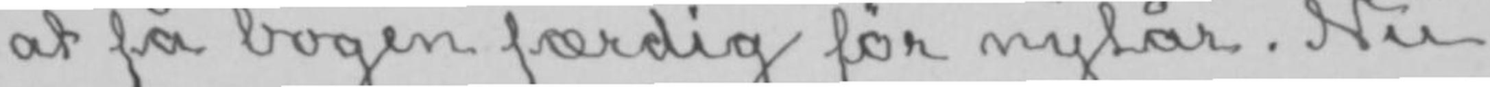at få bogen færdig før nytår. Nu
